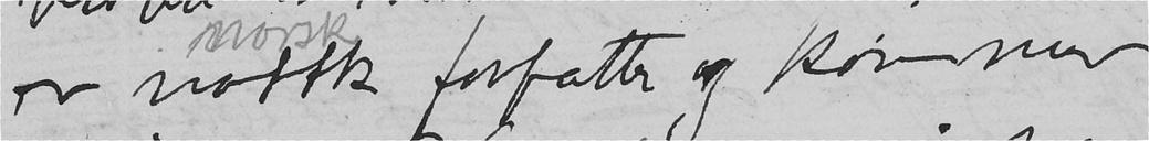er noksk forfatter og kommer
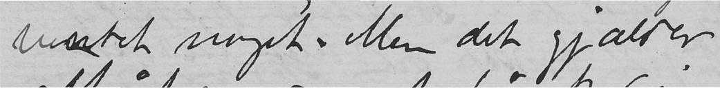ventet noget. Men det gjælder
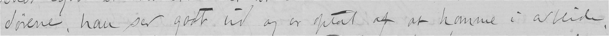dørene, han ser godt ud og er optat af at komme i arbeide,
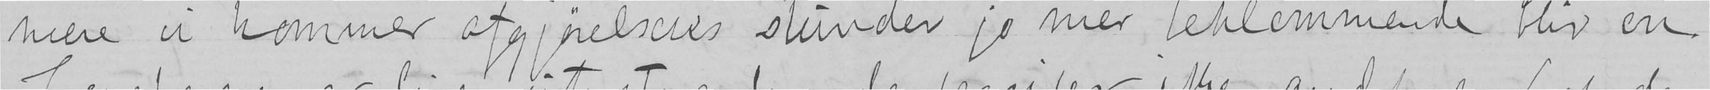mere vi kommer afgjørelsenes stunder jo mer beklemmende blir en.
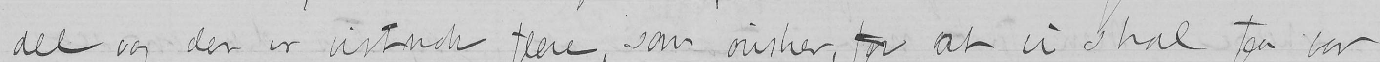del og der er vistnok flere, som ønsker, for at vi skal faa vor
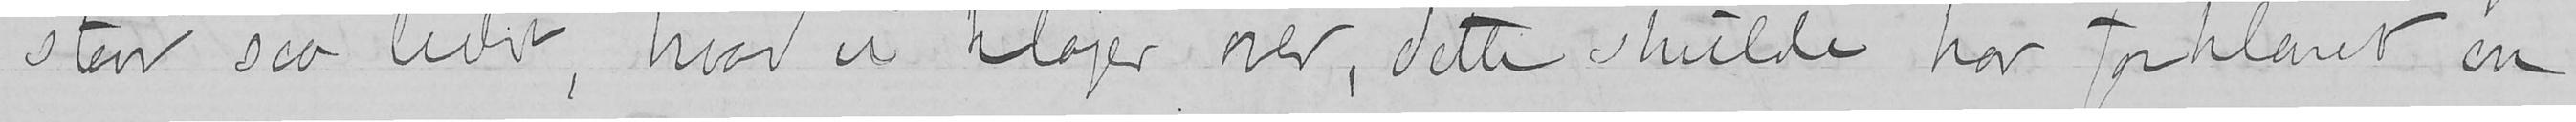staar saa lidet, hvad vi klager over, dette skulde ha forklaret en
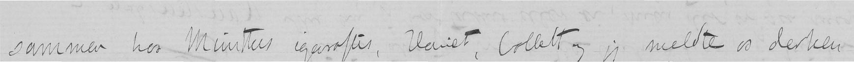sammen hos Munthes igaaraftes, Harriet, Collett og jeg meldte os derhen
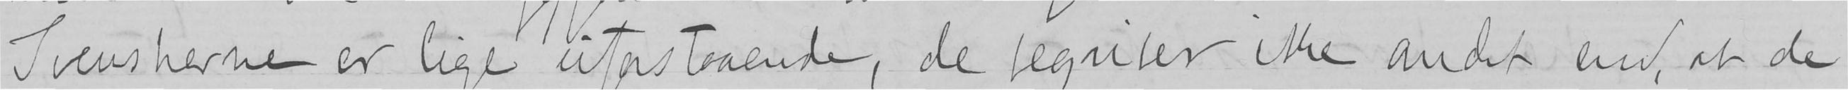Svenskerne er lige uforstaaende, de begriber ikke andet end, at de
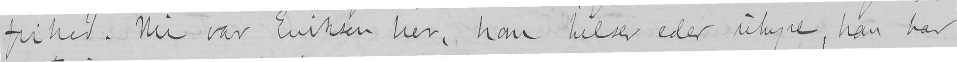frihed. Nu var Eriksen her, han hilser eder uhyre, han har
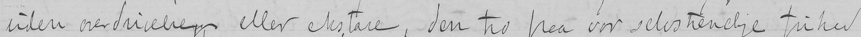uden overdrivelser, eller ekstace, den tro paa vor selvstendige frihed
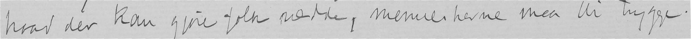hvad der kan gjøre folk rædde, menneskerne maa bli trygge.
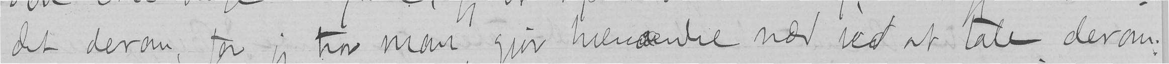det derom, for jeg tror man gjør hverandre ræd ved at tale derom.
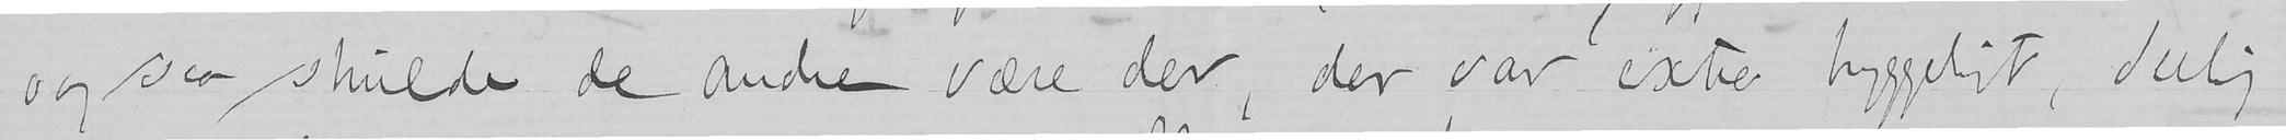ogsaa skulde de andre være der, der var extra hyggeligt, deilig
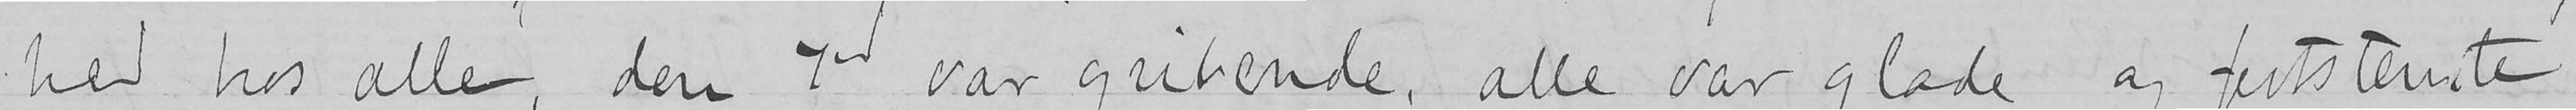hed hos alle, den 7d var gribende, alle var glade og feststemte
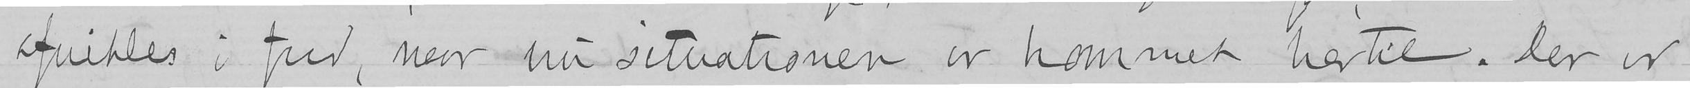afvikles i fred, naar nu situationen er kommet hertil. Der er
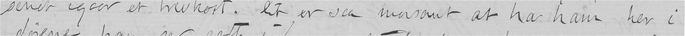sendt igaar et brevkort. Det er saa morsomt at ha ham her i
